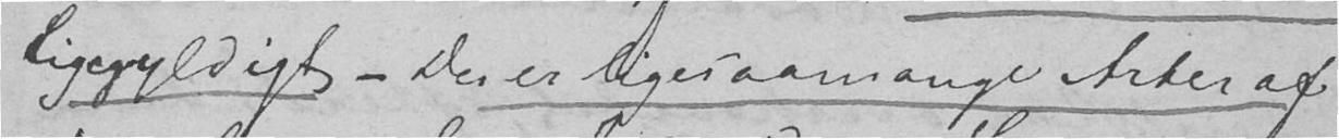ligegyldigt - Der er ligesaamange Arter af
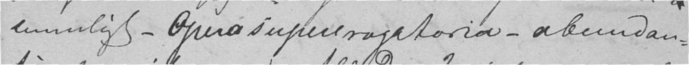umulig – Opersuperagter - abudan-
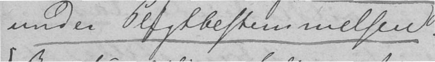under Pligtbestemmelsen.
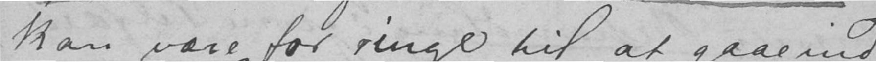kan være for ringe til at gaae ind
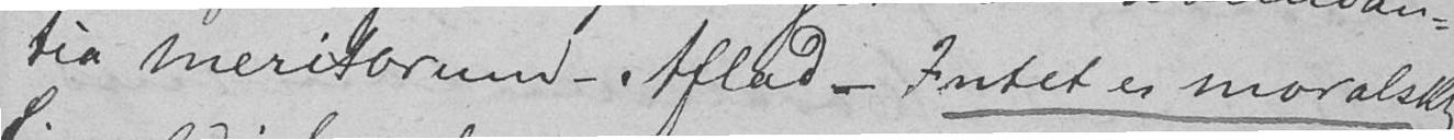tia meritorum – Aflad - Intet er moralskt
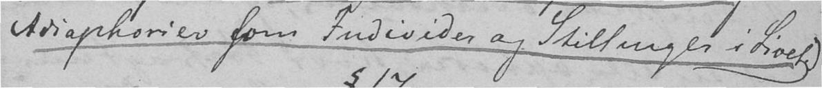Adiaphorier som Individer og Stillinger i Livet).
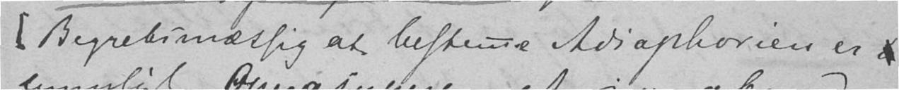Begrebsmæssig at bestemme Adiaphorien er
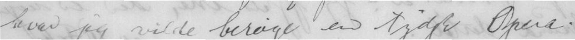hvor jeg vilde besøge en tydsk Opera-
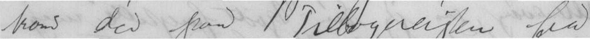kom der paa Tilbagereisen fra
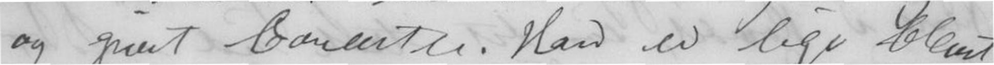og givet Concerter. Han er lige blivet
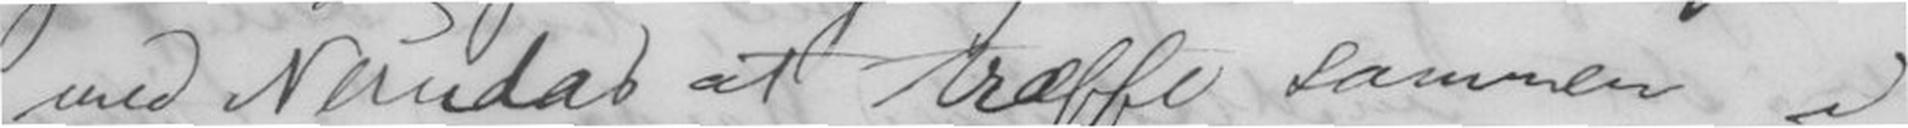med Nerudas at træffe sammen i
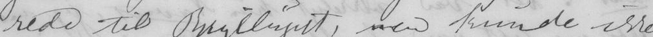rede til Bryllupet, men kunde ikke
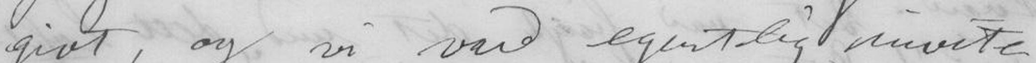givt, og vi vare egentlig invite-
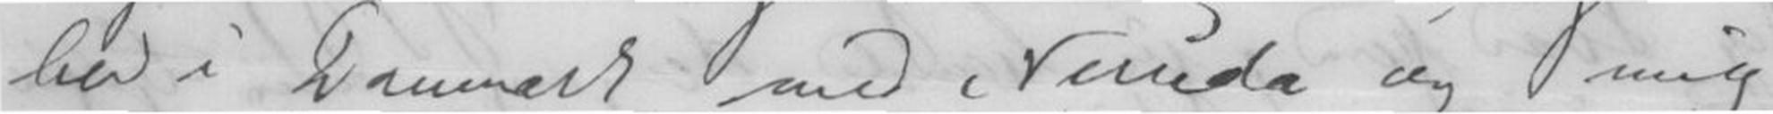her i Danmark med Neruda og mig
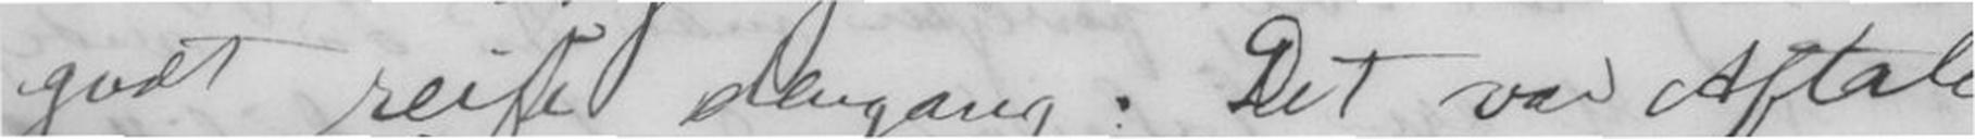godt reise dengang. Det var Aftale
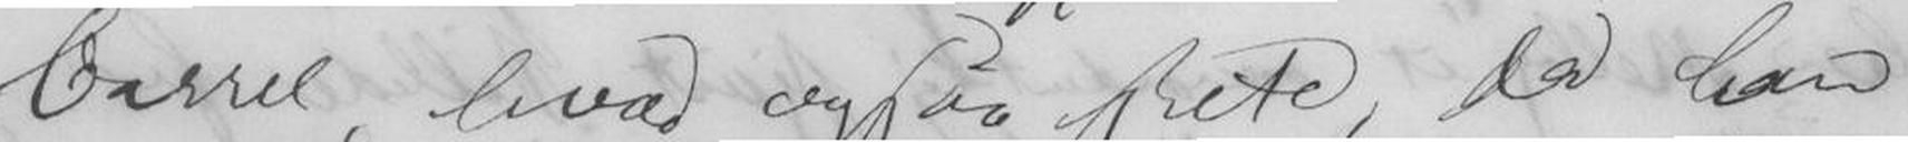Cassel, hvad ogsaa skete, da han
